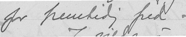for fremtidig fred.
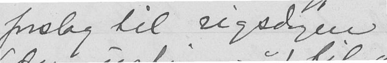forslag til rigsdagen
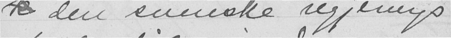k den svenske regjerings
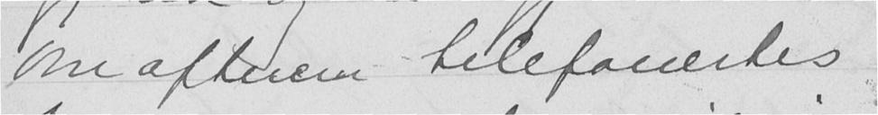Om aftenen telefonertes
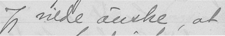Jeg vilde ønske at
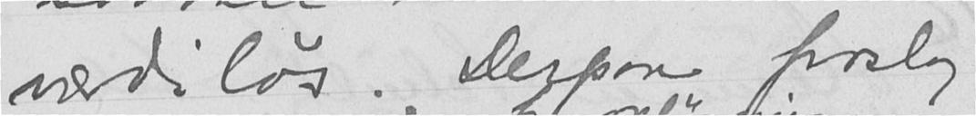verdiløs. Derpaa forslag
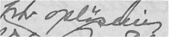for opløsning
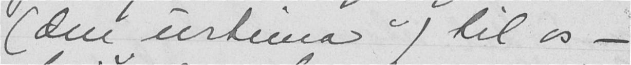(den "urtima") til os -
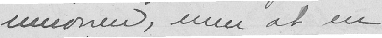unionen, men at en
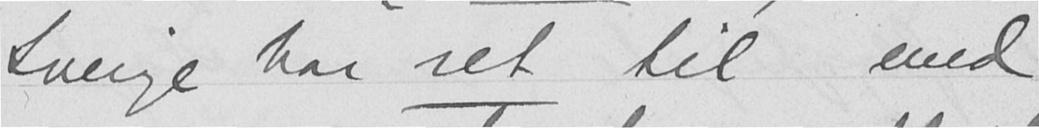Sverige har ret til med
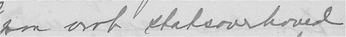paa vort statsoverhoved
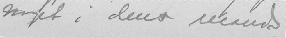noget i dens mands
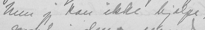Men jeg kan ikke hjælpe,
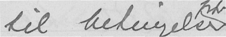til betingelser
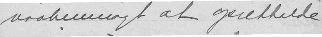vaabenmagt at opretholde
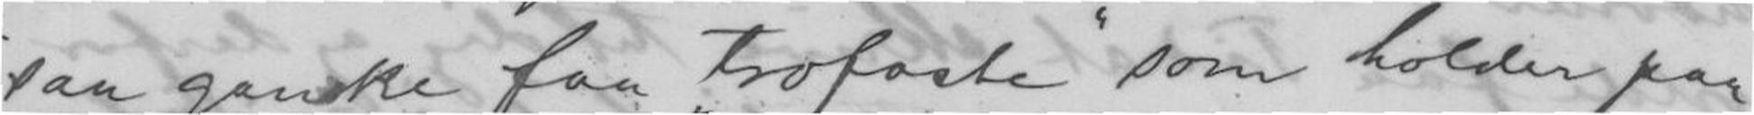saa ganske faa trofaste som holder paa
